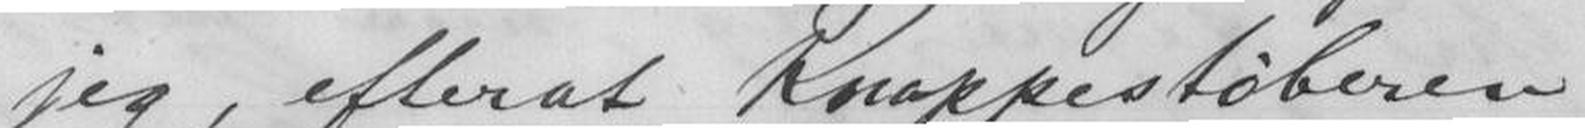jeg, efterat Knappestøberen
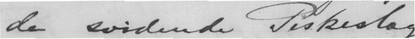de svidende Piskeslag,
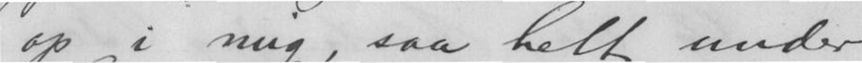op i mig, saa helt under
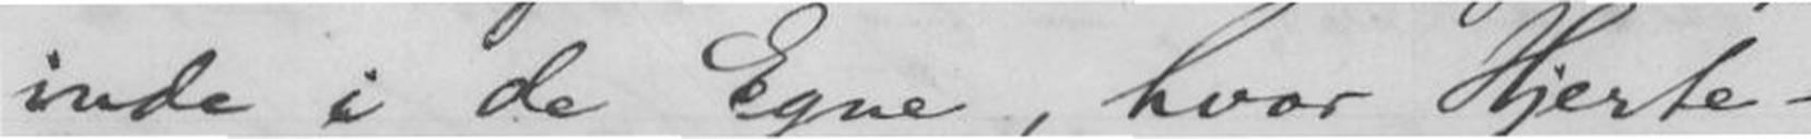inde i de Egne, hvor Hjerte-
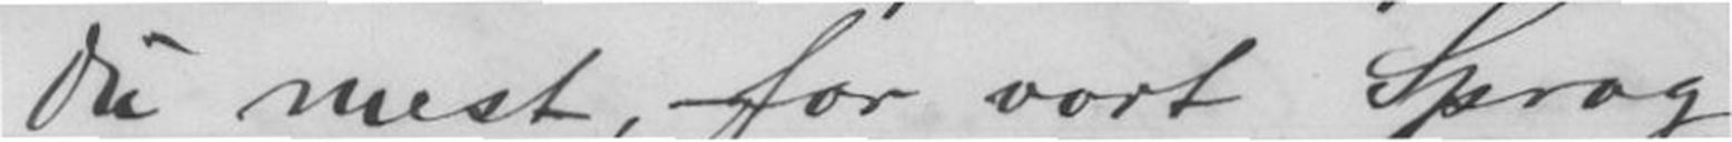Du mest, for vort Sprog
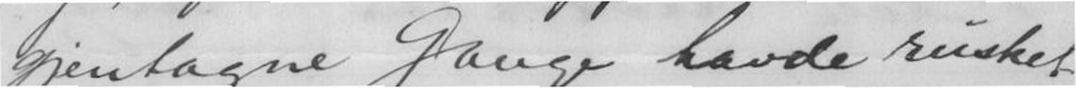gjentagne Gange havde rusket
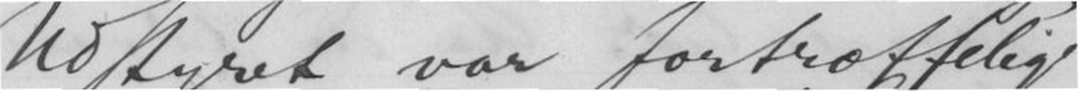Udstyret var fortræffelig,
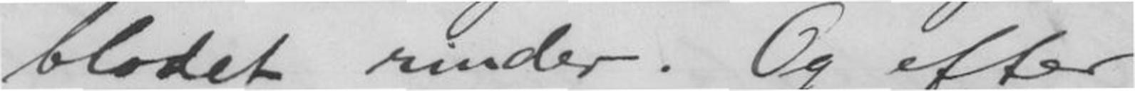blodet rinder. Og efter
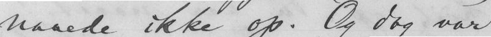naaede ikke op. Og dog var
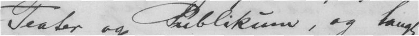Teater og Publikum, og langt
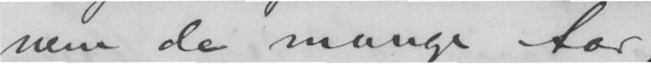nem de mange Aar, -
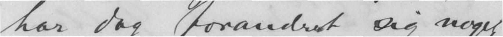har dog forandret sig noget,
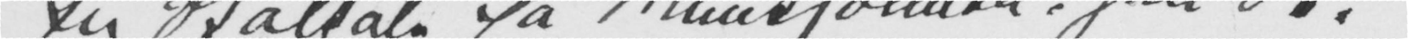En Skåltale på Munkholmen. Juli 87.
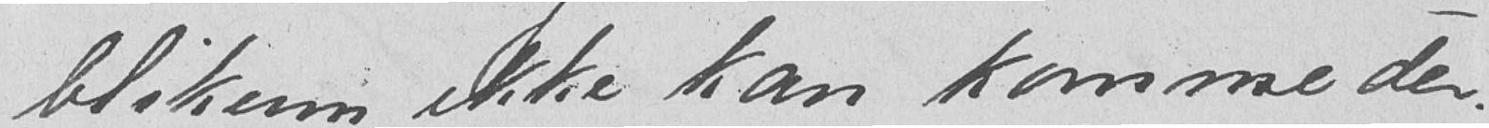blikum ikke kan komme der.
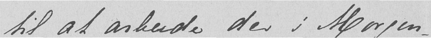til at arbeide der i Morgen-
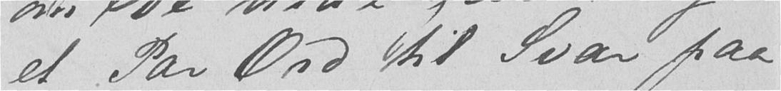et Par Ord til Svar paa
